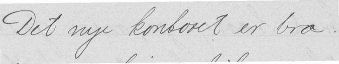Det nye kontoret er bra.
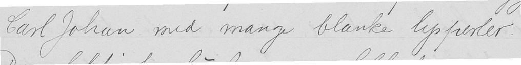Carl Johan med mange blanke lysperler.
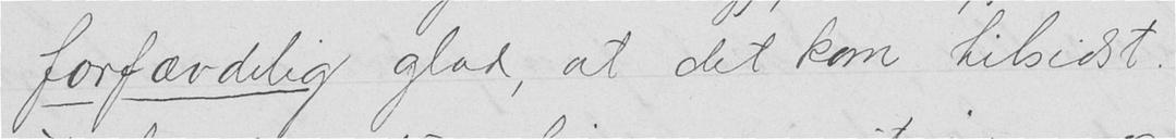forfærdelig glad, at det kom tilsidst.
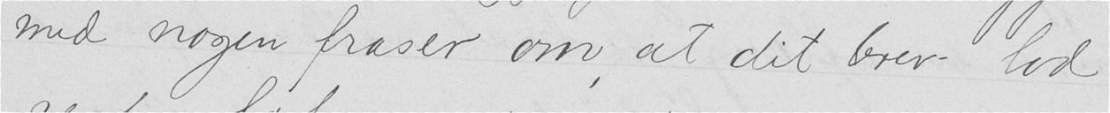med nogen fraser om, at dit brev lod
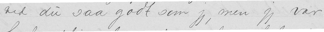ved du saa godt som jeg, men jeg var
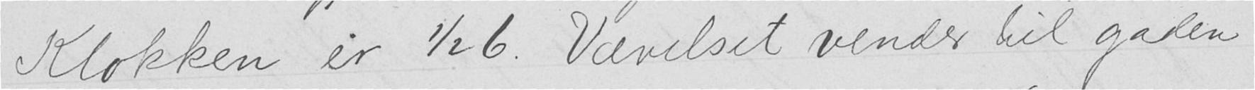Klokken er 1/2 6. Værelset vender til gaden
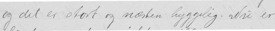og det er stort og næsten hyggelig. Nu er
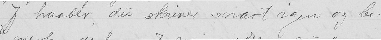Jeg haaber, du skriver snart igen og be-
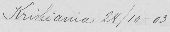Kristiania 28/10 - 03
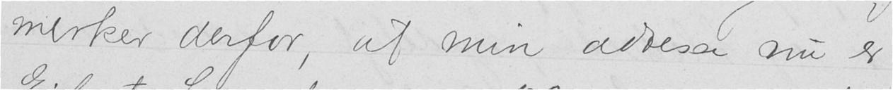merker derfor, at min adresse nu er
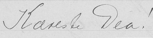Kæreste Dea!
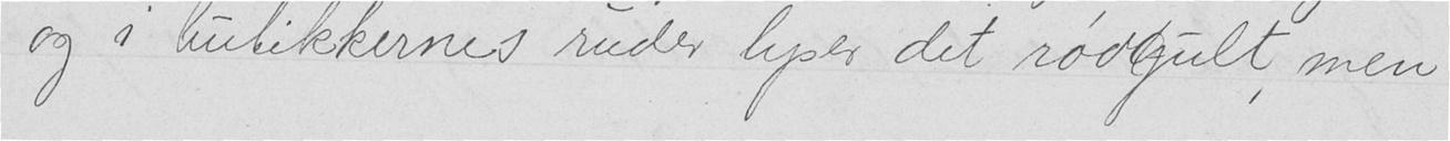og i butikkernes ruder lyser det rødgult, men
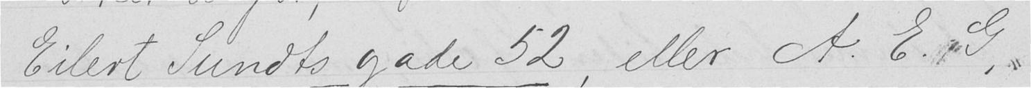Eilert Sundts gade 52, eller A. E. G.,
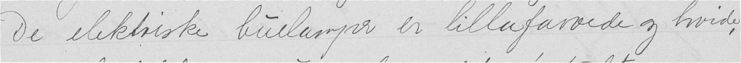De elektriske buelamper er lillafarvede og hvide,
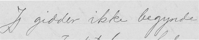Jeg gidder ikke begynde
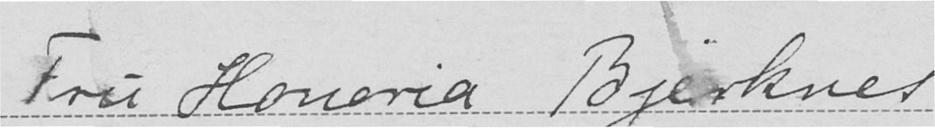Fru Honoria Bjerknes
